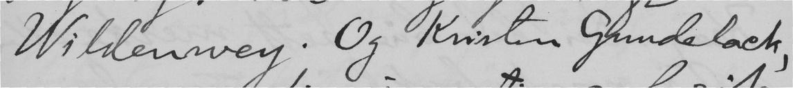Wildenwey. Og Kristen Gundelack,
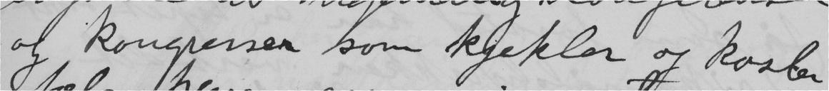og Kongresser som kjekler og koster
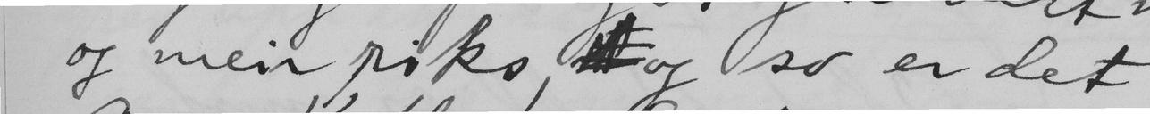og meir riks, og so er det
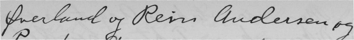Øverland og Rein Andersen og
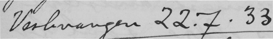Veslevangen 22.7.33
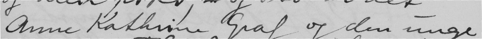Anne Kathrine Gral og den unge
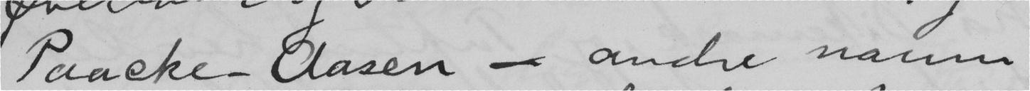Paacke-Aasen – andre namn
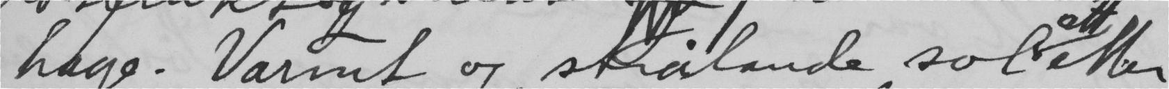hage. Varmt og strålande sol att etter
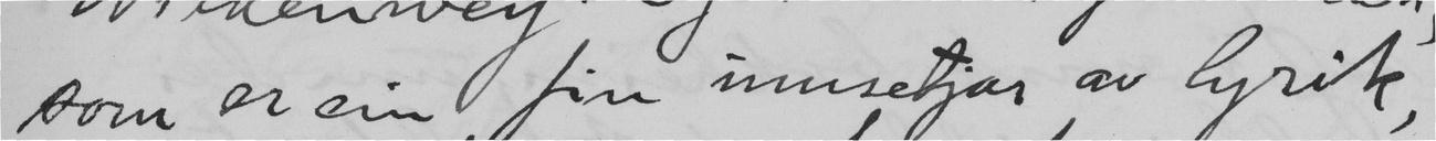som er ein fin umsetjar av lyrik,
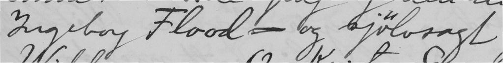Ingeborg Flood – og sjölvsagt
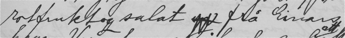rotfrukt og salat  frå Einars
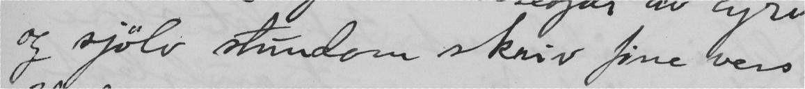og sjölvstmundom skriv fine vers
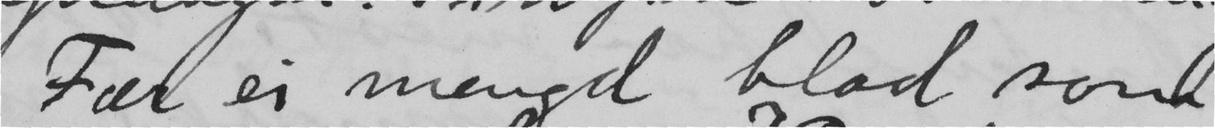Fær ei mengd blad som
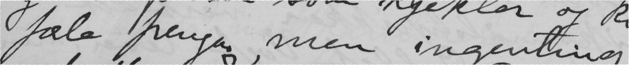fæle pengar men ingenting
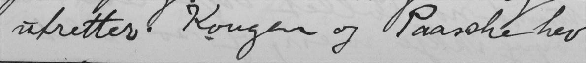utsetter Kongen og Paasche hev
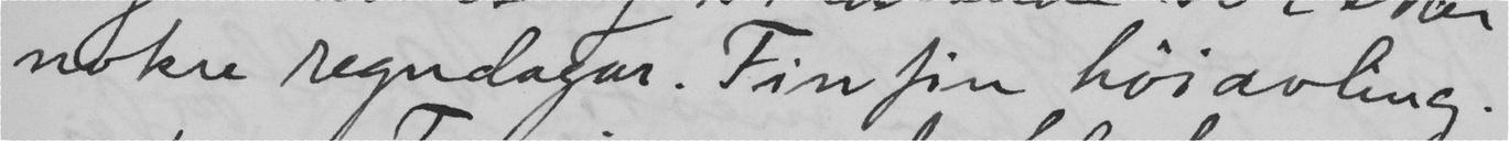nokre regndagar. Fin fin höiavling.
